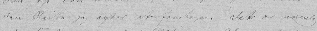den Reise jeg agter at foretage. Det er nemlig
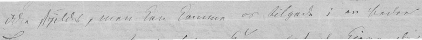ikke spildes, men kan komme os tilgode i en bedre
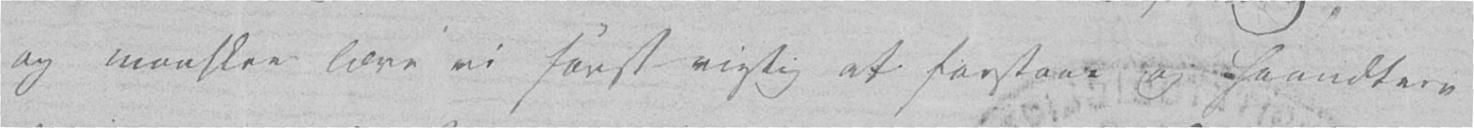og maaskee lære vi først rigtig at forstaae og haandtere
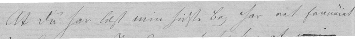At Du har læst min sidste Bog har ret fornøiet
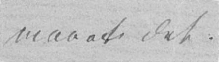maaet det.
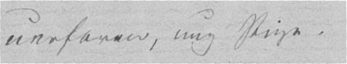uerfaren, ung Pige.
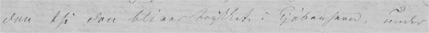den thi den bliver trykket i Kjøbenhavn, under
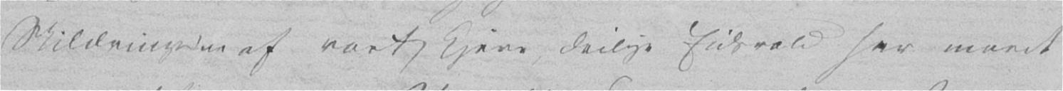Skildringerne af vort kjere, deilige Eidsvold har moret
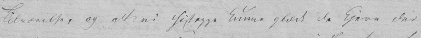Tilværelse, og at vi histoppe kunne glæde de kjere der
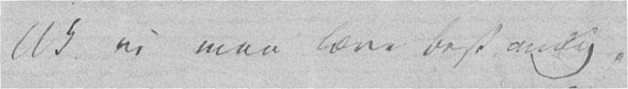Ak vi maa lære bestandig,
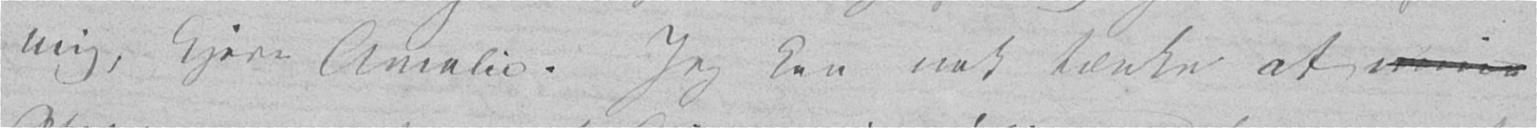mig, kjere Amalie. Jeg kan nok tænke at mine
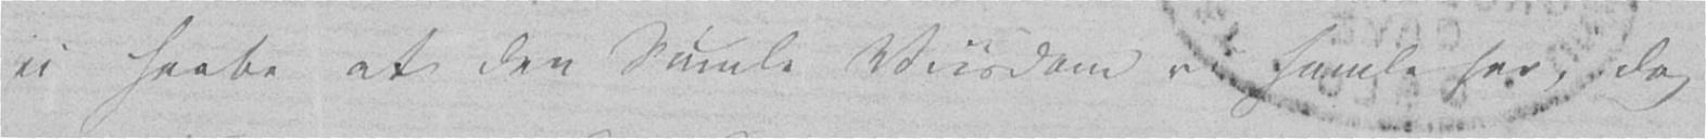vi haabe at den Smule Viisdom vi samle her, dog
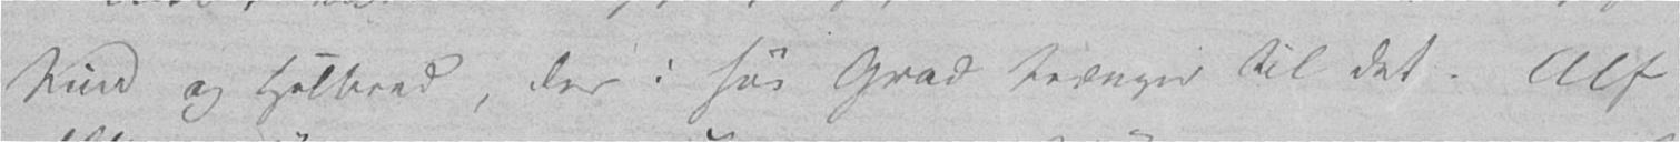Sind og Helbred, der i høi Grad trænger til det. Alf
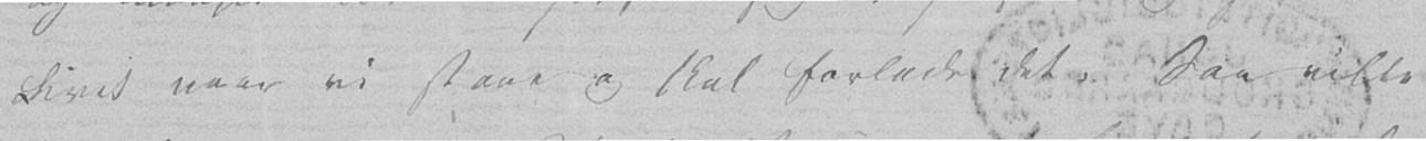Livet naar vi staae og skal forlade det. Saa ville
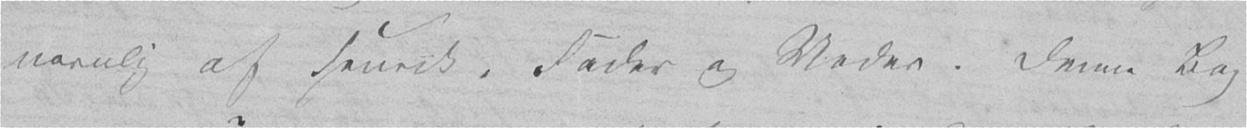navnlig af Henrik, Fader og Moder. Denne Bog
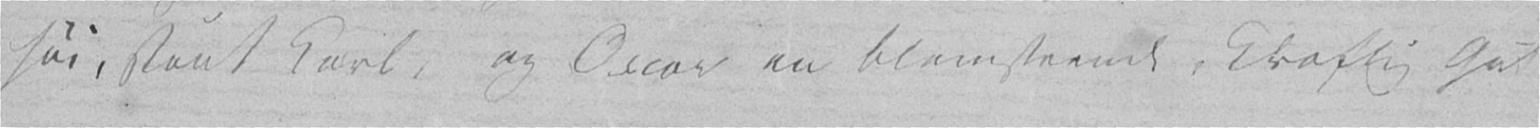høi, staut Karl, og Oscar en blomstrende, kraftig Gut
